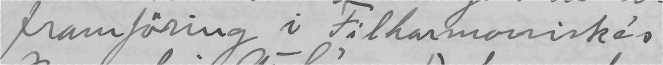framföring i Filharmoniskes
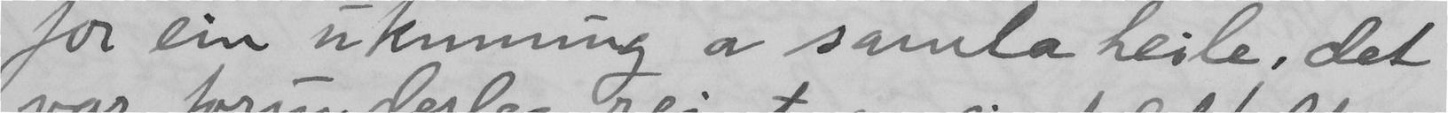for ein ukunning å samla heile; det
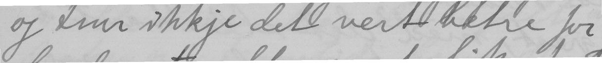og trur ikkje det vert betre for
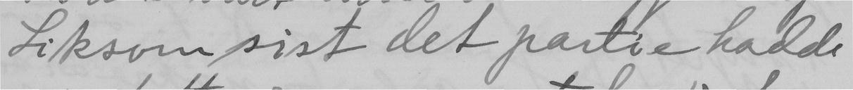Liksom sist det partie hadde
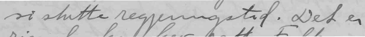si slutte regjeringstid. Det er
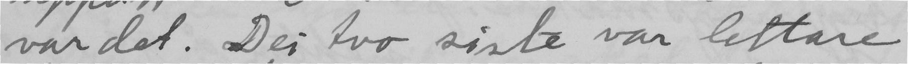var det. Dei tvo siste var lettare
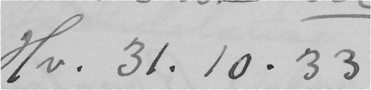Hv. 31.10.33
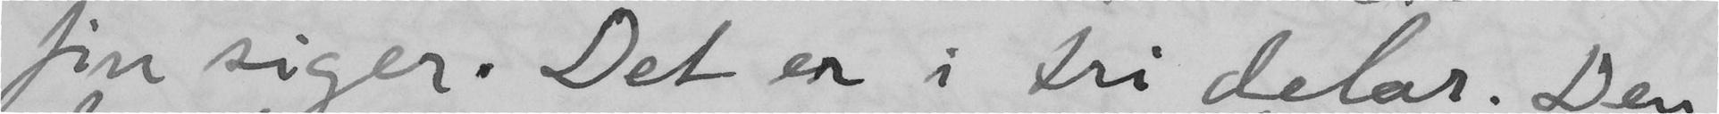fin siger. Det er i tri delar. Den
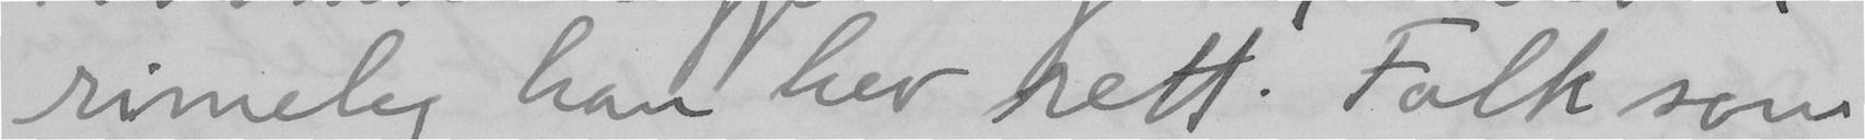rimeleg han hev rett. Folk som
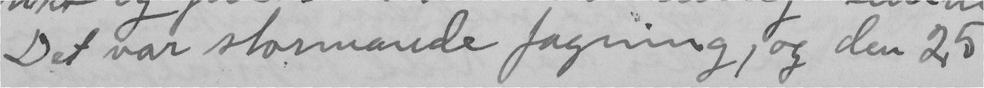Det var stormande fagning, og den 25
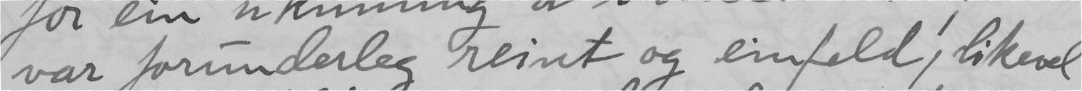var forunderleg reint og einfeld; likevel
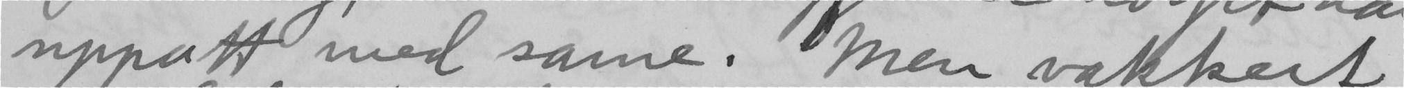uppått med same. Men vakkert
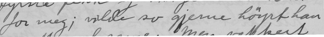for meg; vilde so gjerne höyrt han
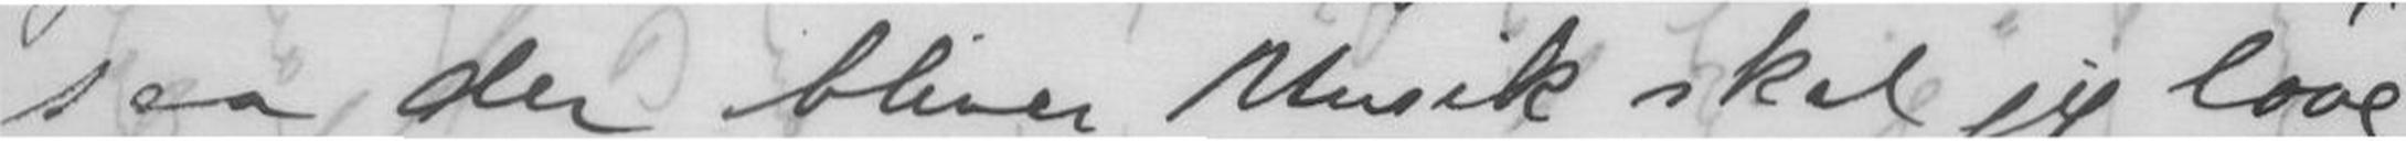saa der bliver Musik skal jeg love.
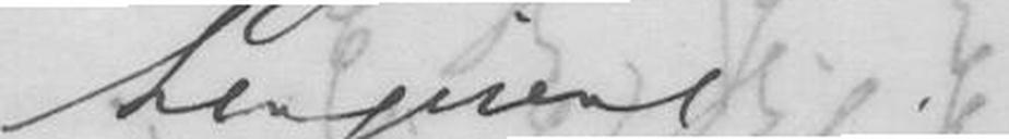hengivne
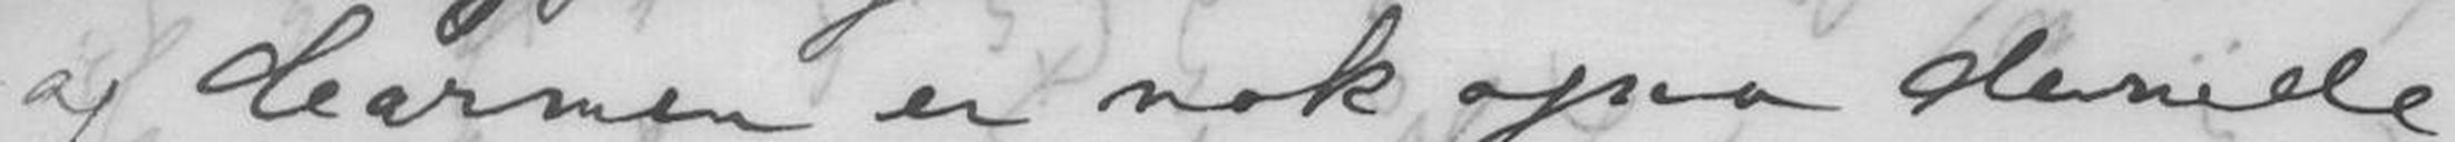og Carmen er nok ogsaa dernede,
